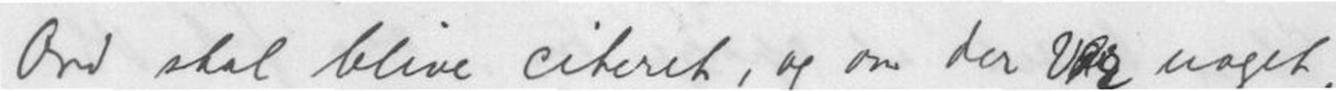Ord skal blive citeret, og om der var noget,
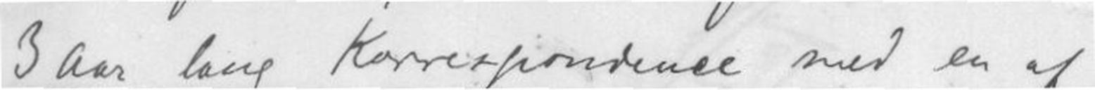3 Aar lang Korresponderes med en af
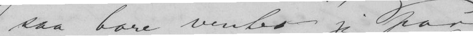saa bare venter jeg paa
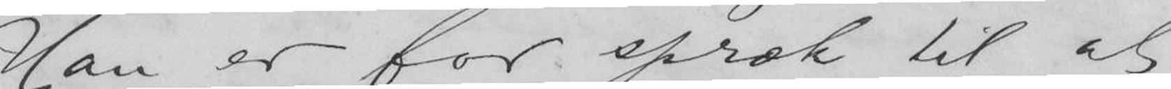Han er for spræk til at
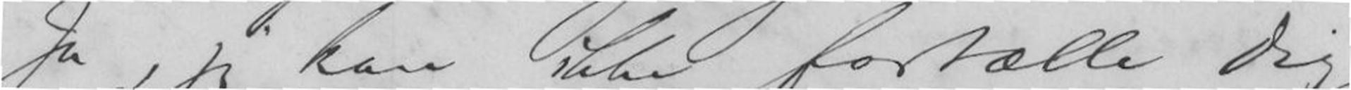Ja, jeg kan ikke fortælle dig,
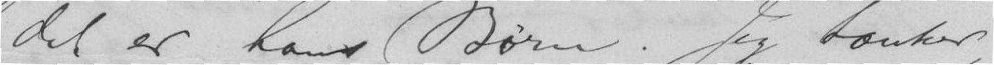det er hans Børn. Jeg tænker,
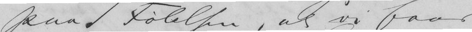paa Følelsen, at vi faar
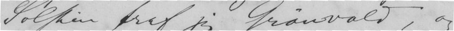Solskin traf jeg Grønvold, og
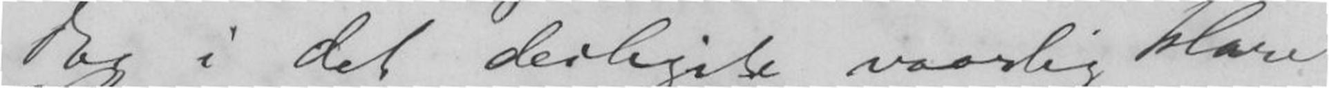dag i det deiligste vaarlig klare
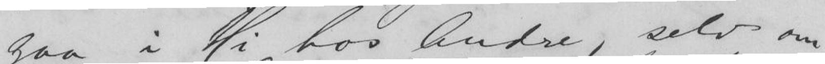gaa i Hi hos Andre, selv om
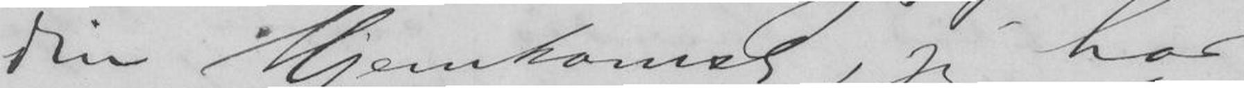din Hjemkomst, jeg har
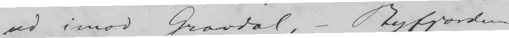ud imod Gravdal, - Byfjorden
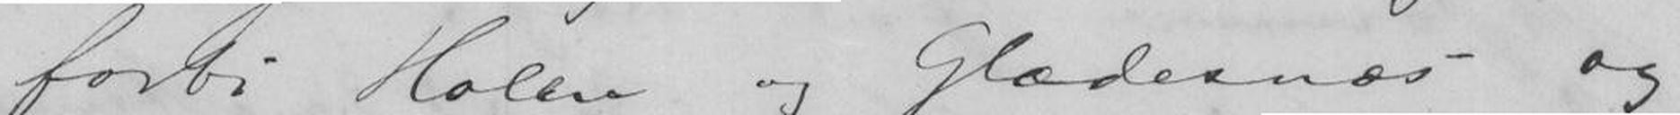forbi Holen og Glædesnæs og
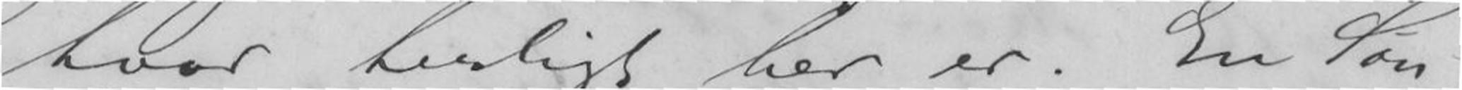hvor herligt her er. En Søn-
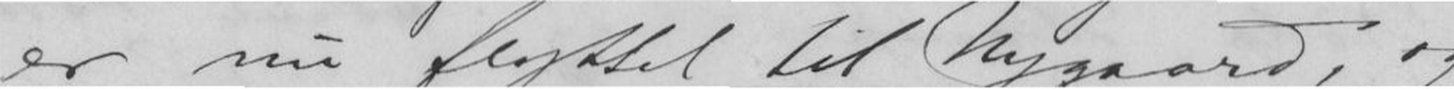er nu flyttet til Nygaard, og
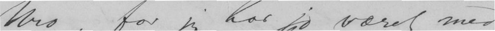Uro, for jeg har jo været med
